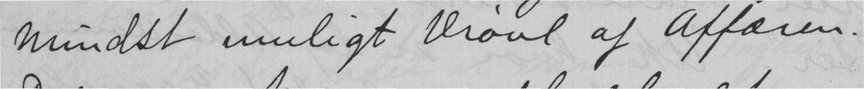mindst muligt Vrøvl af Affæren.
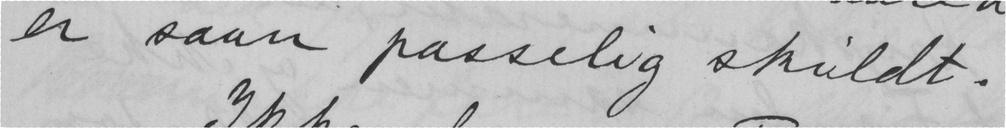er saan passelig skildt.
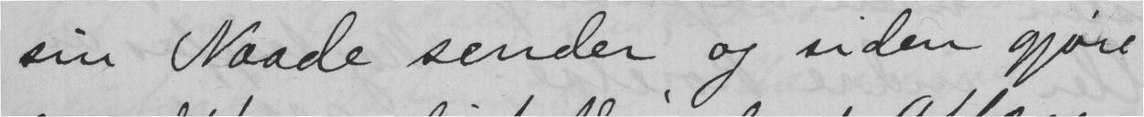sin Naade sender og siden gjøre
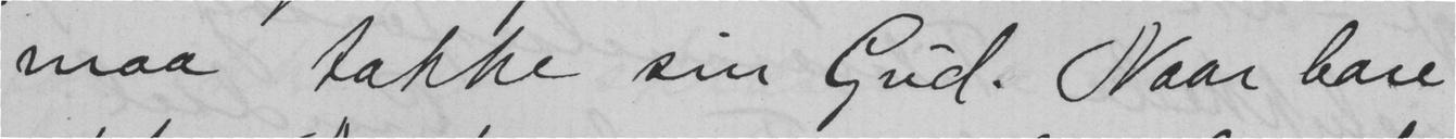maa takke sin Gud. Naar bare
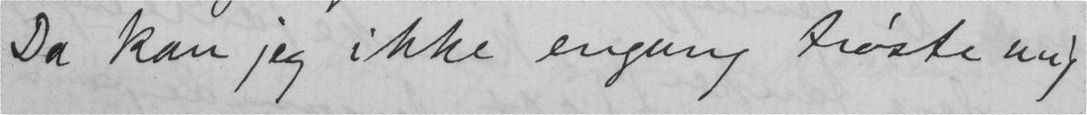Da kan jeg ikke engang trøste mig
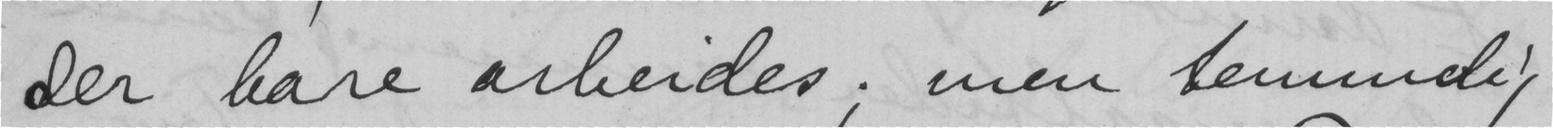der bare arbeides; men temmelig
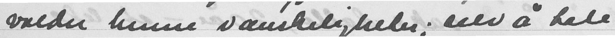volder henne vanskeligheter; selv å tale
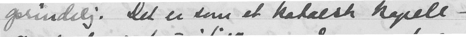oprindelig. Det er som et katolsk kapell -
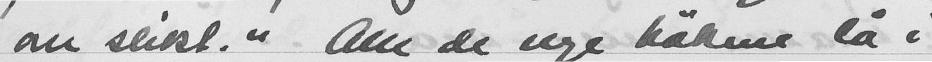om slikt." Alle de nye bøkene lå i
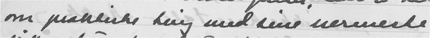om praktiske ting med sine nermeste
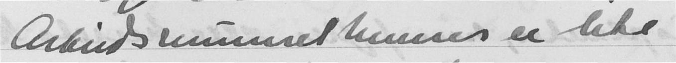Arbeidsrummet hennes er lite
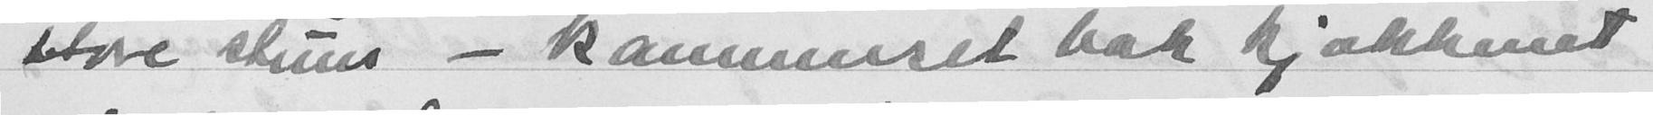store stuen - kammerset bak kjøkkenet
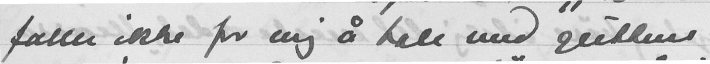faller ikke for mig å tale med gutten
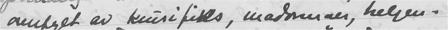overfylt av krusifiks, madonnaer, helgen-
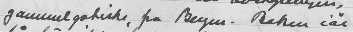gammelgotiske, fra Bergen. Boken iår
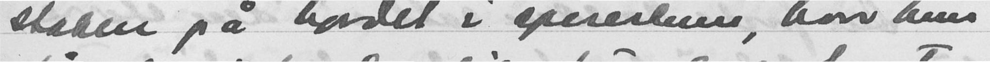stabler på bordet i spisestuen, hvor hun
